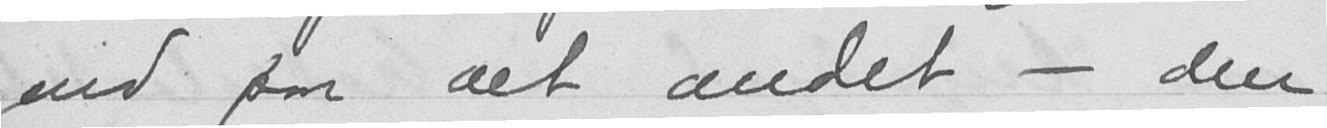end paa alt andet - den
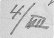4/VII
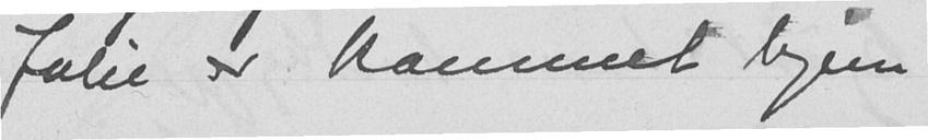Julie er kommet hjem
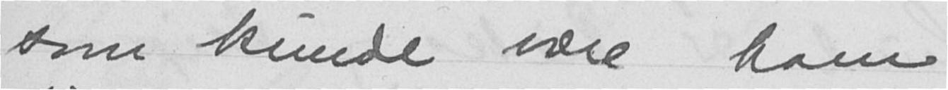som kunde være ham
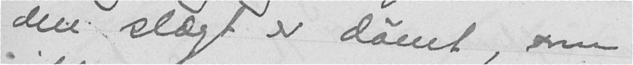den slægt er dømt, som
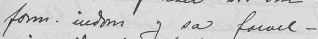form. indom og sa farvel -
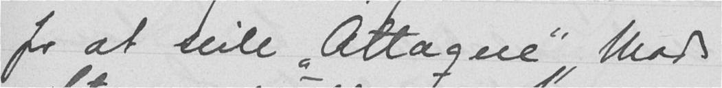for at seile "Attaque", Mads
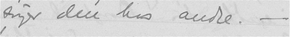søger den hos andre. -
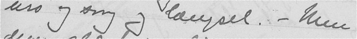uro og sorg og længsel. - Men
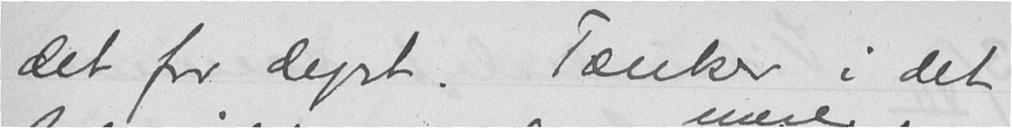det for dyrt. Tænker i det
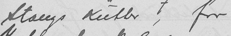Stangs kutter, for
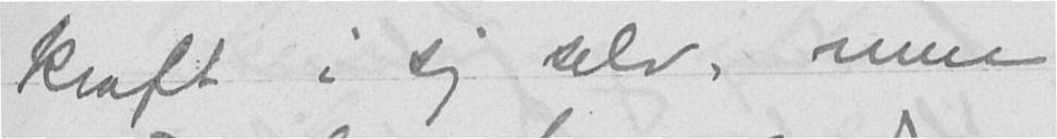kraft i sig selv, men
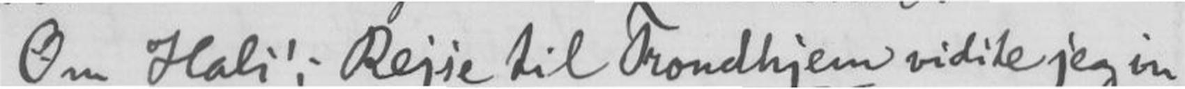Om Hals, -Rejse til Trondhjem vidste jeg in-
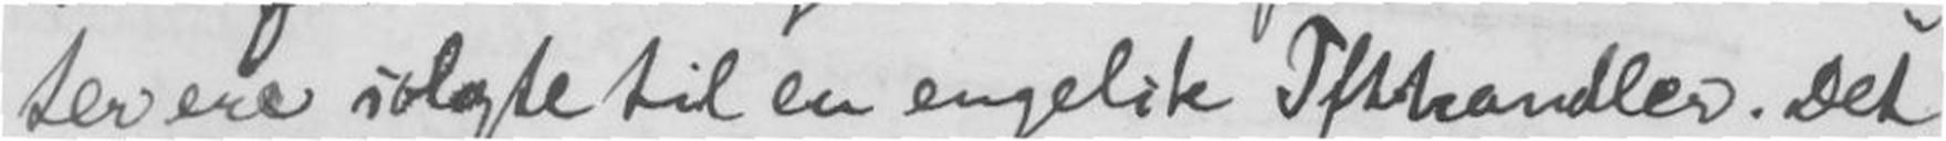ter ere solgte til en engelsk Tfthandler. Det
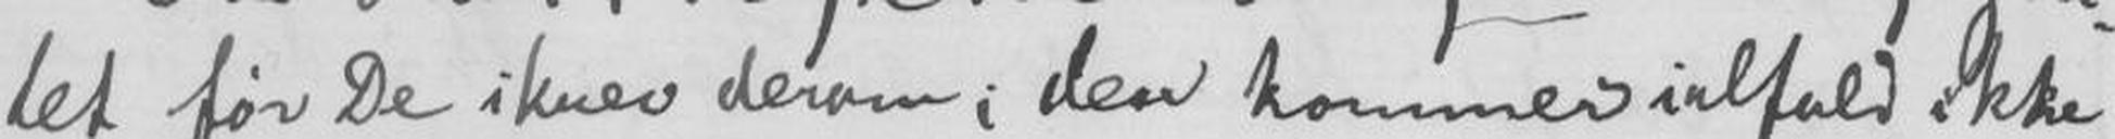tet før De skrev derom; den kommer ialtfald ikke
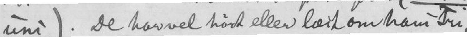uns). De har vel hørt eller læst om hans Tri
